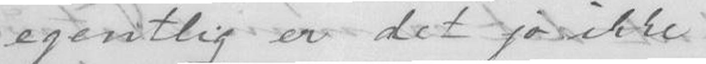egentlig er det jo ikke
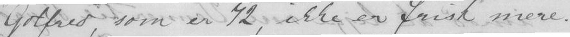Gotfred, som er 72, ikke er frisk mere.
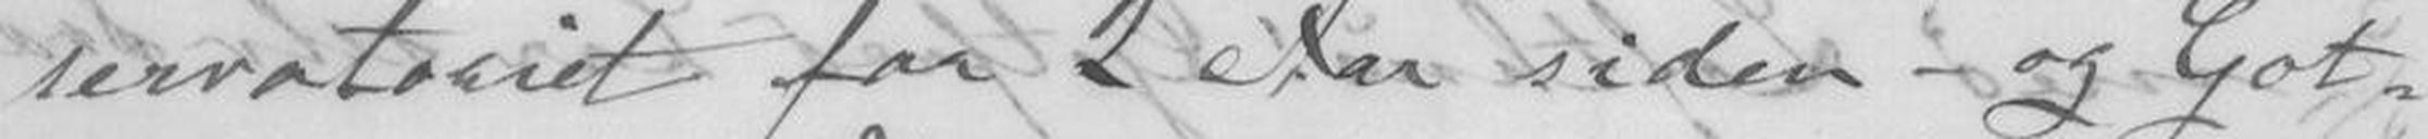servatoriet for 2 Aar siden - og Got-
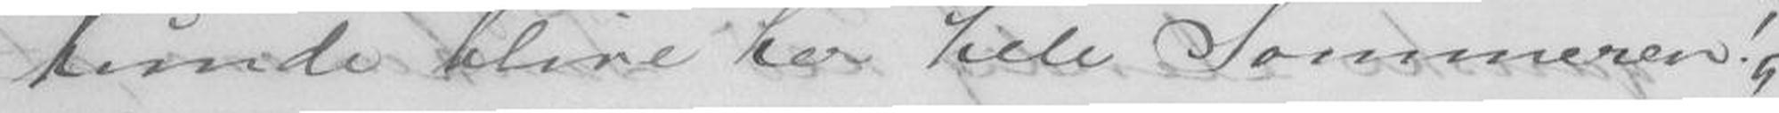kunde blive her hele Sommeren!
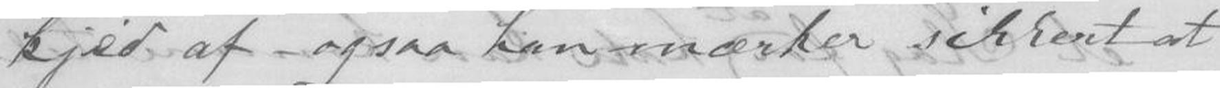kjed af - ogsaa han mærker sikkert at
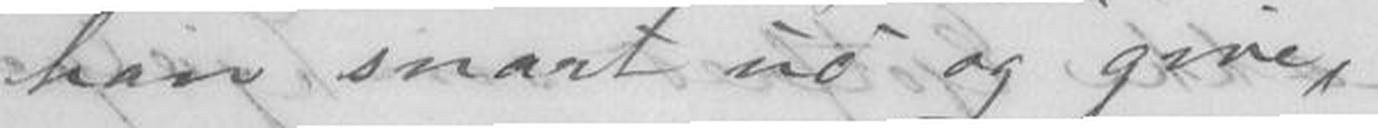han snart ud og give,
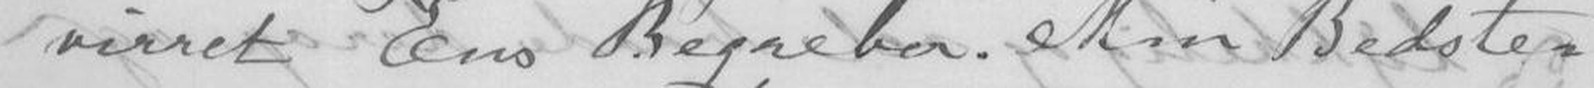virret Ens Begreber. Min Bedste-
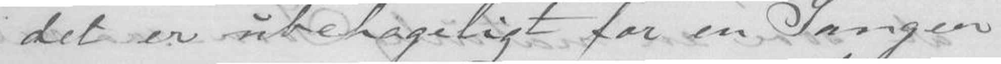det er ubehageligt for en Sanger
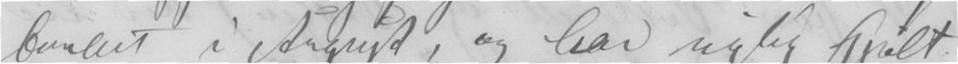Concert i August, og har nylig spilt
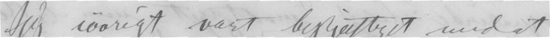jeg iøvrigt vært beskjæftiget med at
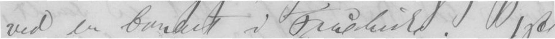ved en Concert i Frankrike; 1ste
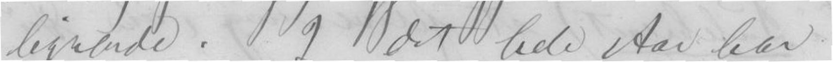lignende. I det hele Aar har
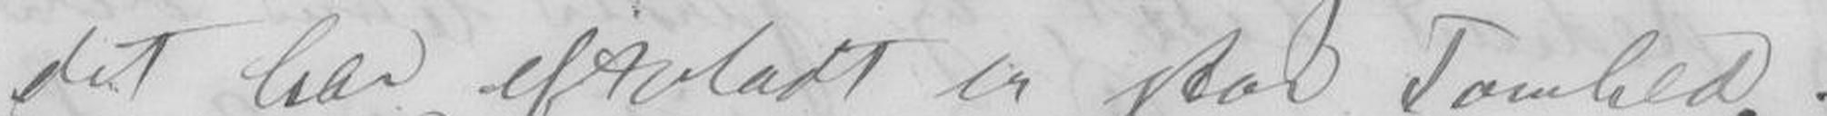det har efterladt en stor Tomhed.
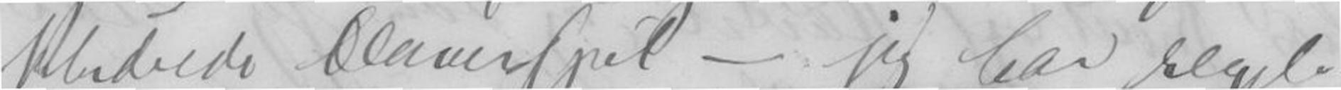kludrede Claverspil - jeg har regel-
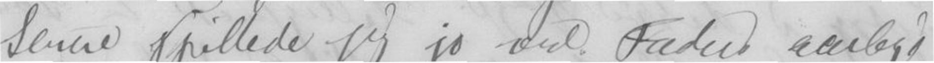Senere spillede jeg jo ved Faders aarlige
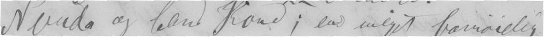Neruda og hans Kone; en meget fornøielig
